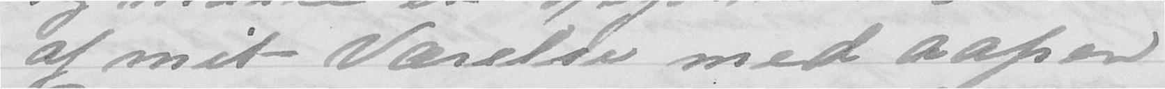at mit Værelse med aapen
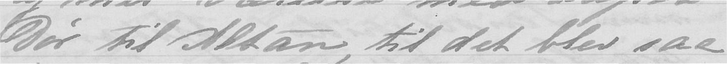Dør til Altan, til det blev saa
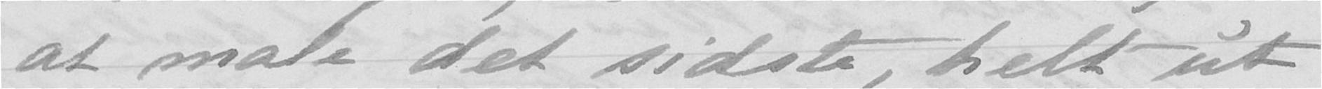at male det sidste, helt ut
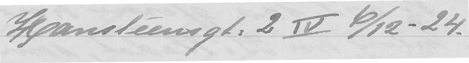Hansteensgt: 2 IV 6/12-24
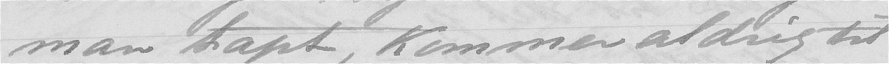man tapt, kommer aldrig til
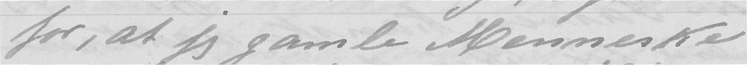for, at jeg gamle Menneske
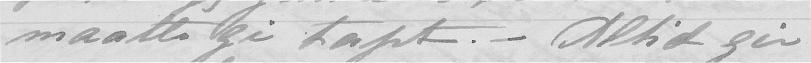maatte gi tapt. Altid gir
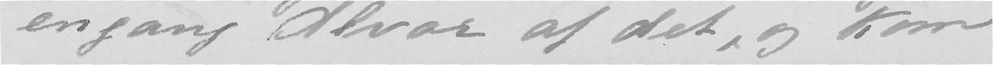engang Alvor af det, og kom
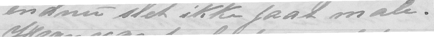endnu slet ikke faat male.
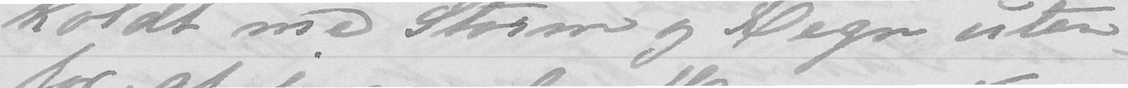Koldt med Storm og Regn uten-
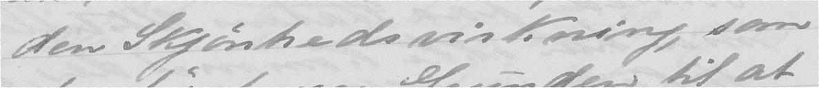den Skjønhedsvirkning, som
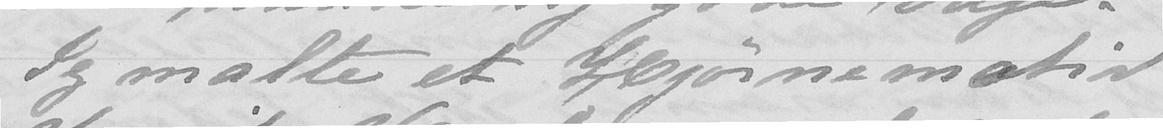Jeg malte et Hjørnemotiv
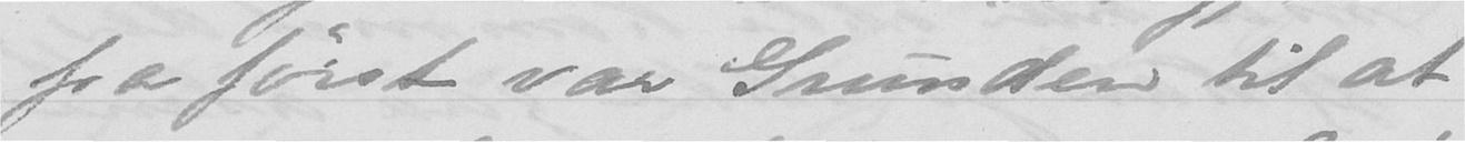fra først var Grunden til at
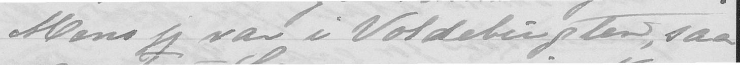Mens jeg var i Voldebugten, saa
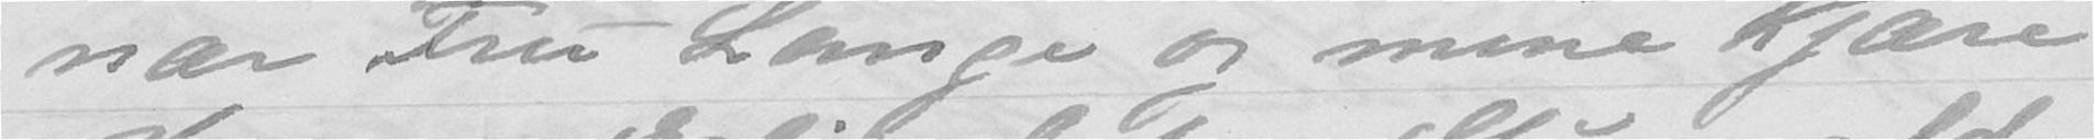nær Fru Lange og mine kjære
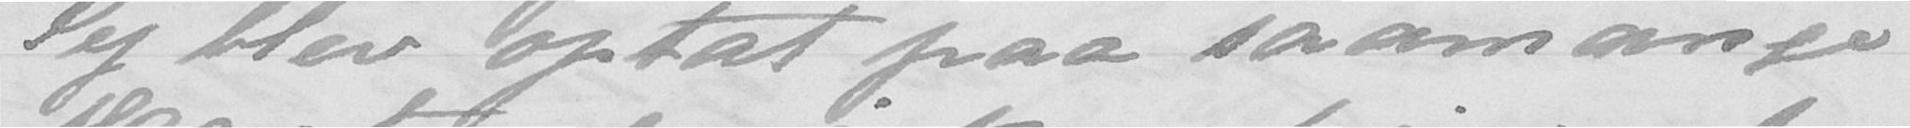Jeg blev optat paa saamange
